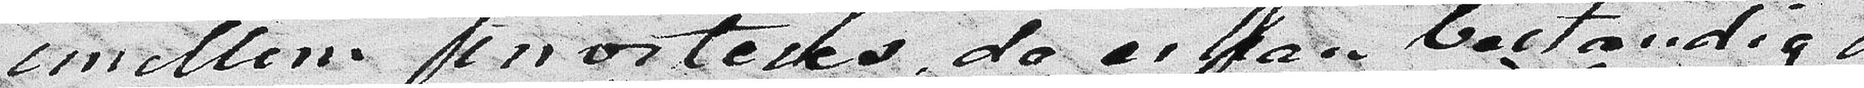imellem inviteres, da er han bastandig
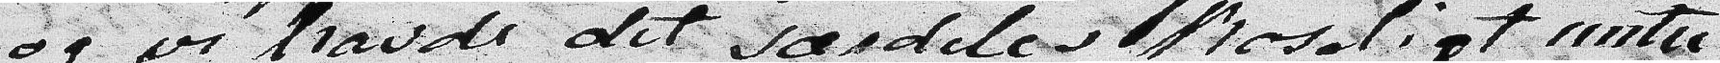og vi havde det særdeles koseligt unter
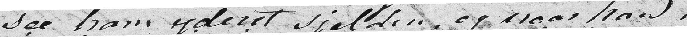see ham yderst sjelden, og naar han
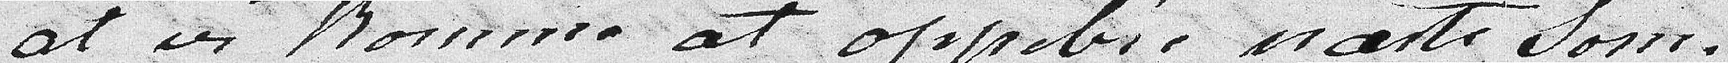at vi komme at oppebie næste Som-
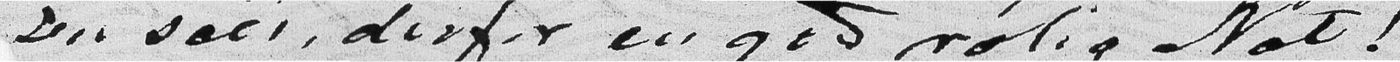Du seer, derfor en god rolig Nat!
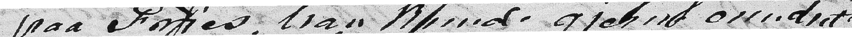pa Fries, han kunde gjerne erindret
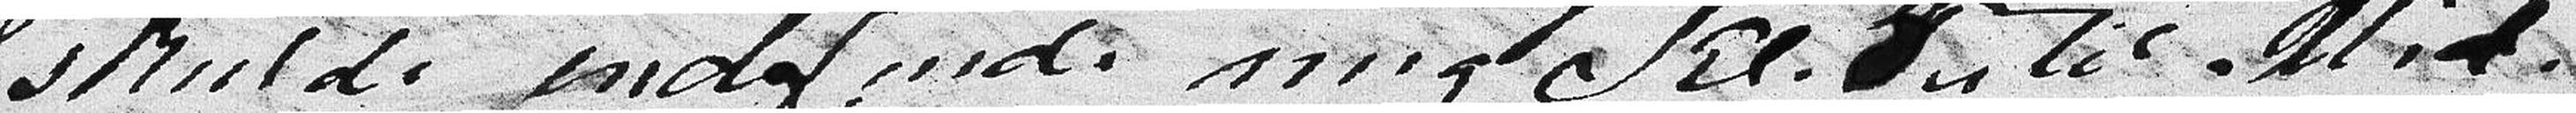skulde infinde mig Kl. Tre til Mid-
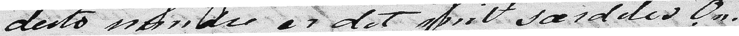desto mindre er det mit særdeles Øn-
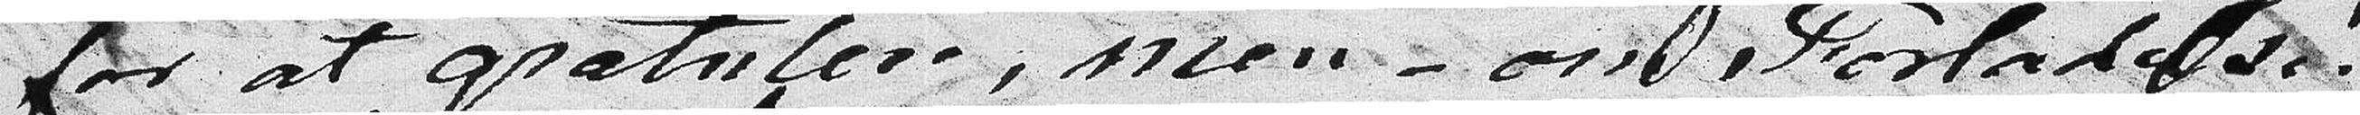for at gratulere, men - om Forladelse!
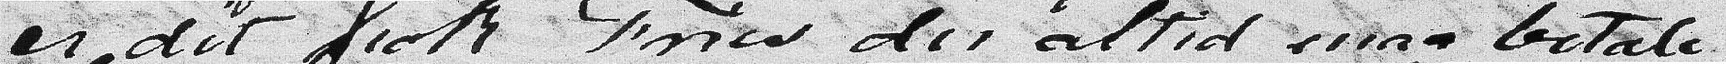er det nok Fries der altid maa betale
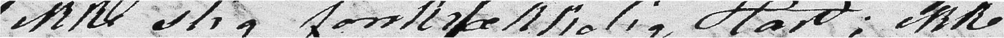ikke slig forskrækkelig Hast; ikke
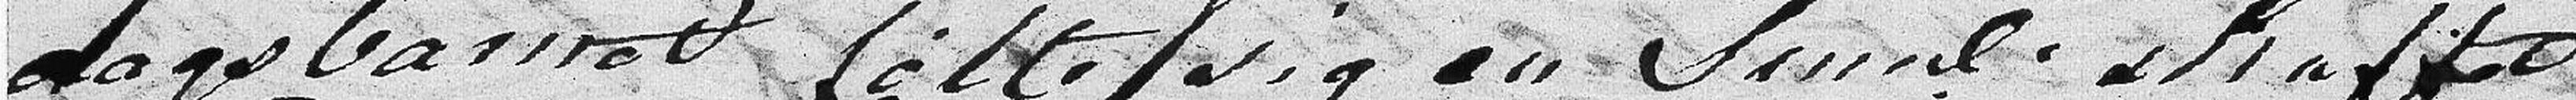dagsbarnet følte sig en Smule skuffet
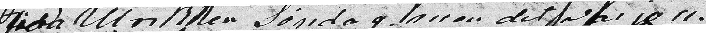paa Ulrikken Søndag, men det var jo u-
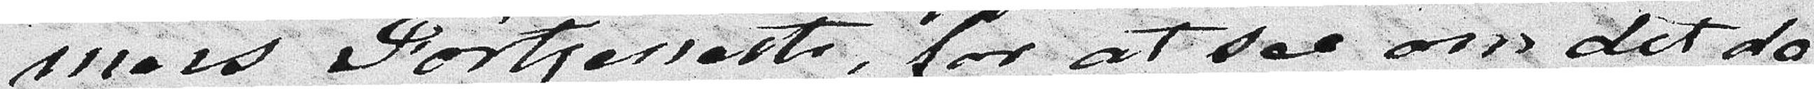mers Fortjeneste, for at see om det da
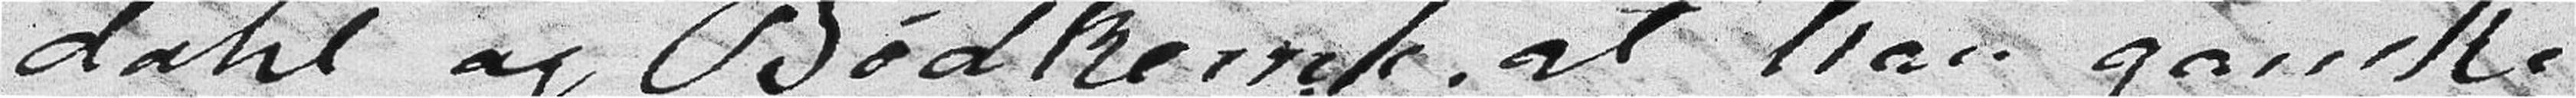dahl og Bødkerne, at han ganske
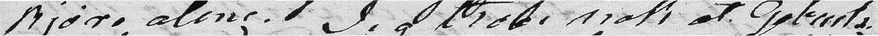kjøre alene. Jeg troer nok at Geburts-
